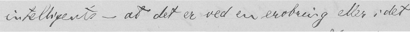intelligents - at det er ved en erobring eller i det
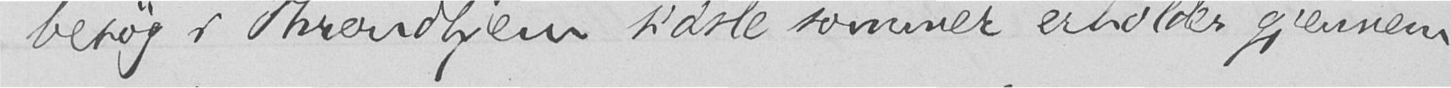besøg i Throndhjem sidste sommer erholder gjennem
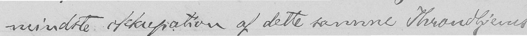mindste okkupation af dette samme Throndhjems
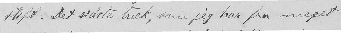stift. Det sidste træk, som jeg har fra meget
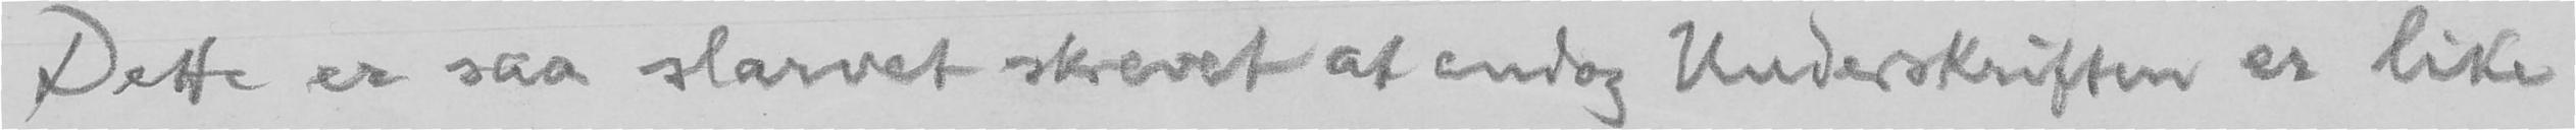Dette er saa slarvet skrevet at endog Underskriften er like
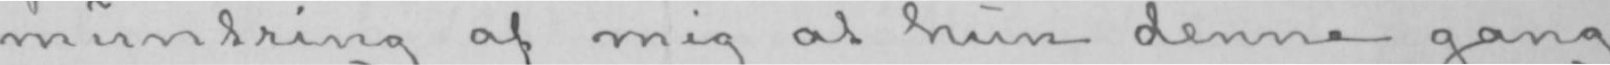muntring af mig at hun denne gang
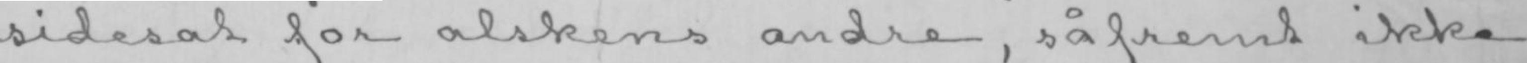sidesat for alskens andre, såfremt ikke
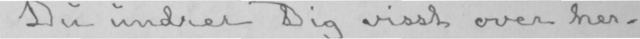Du undrer Dig visst over her-
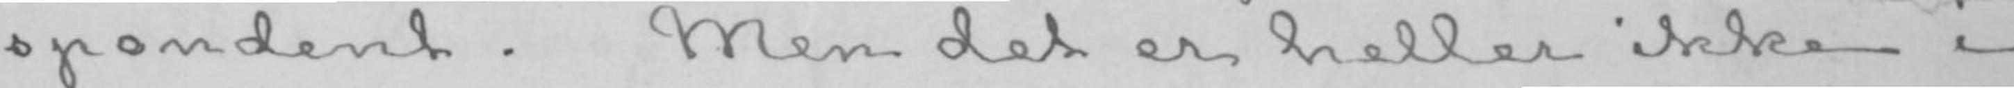spondent. Men det er heller ikke i
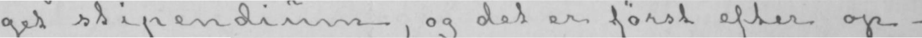get stipendium, og det er først efter op-
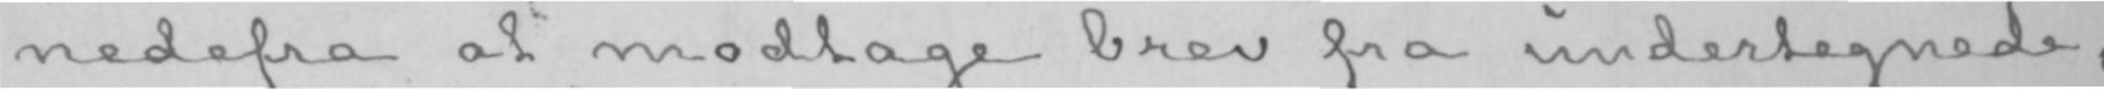nedefra at modtage brev fra undertegnede,
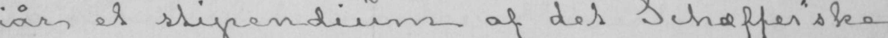iår et stipendium af det Schæffer&rapo;ske
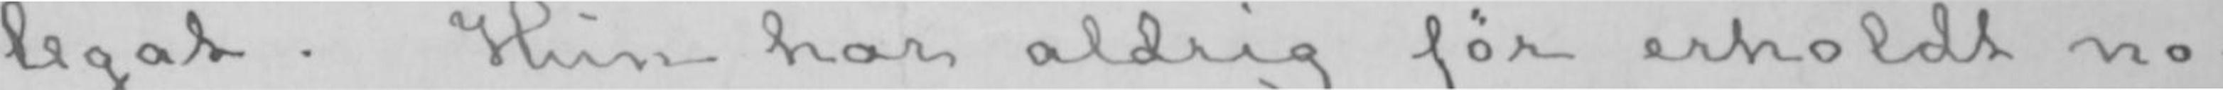legat. Hun har aldrig før erholdt no-
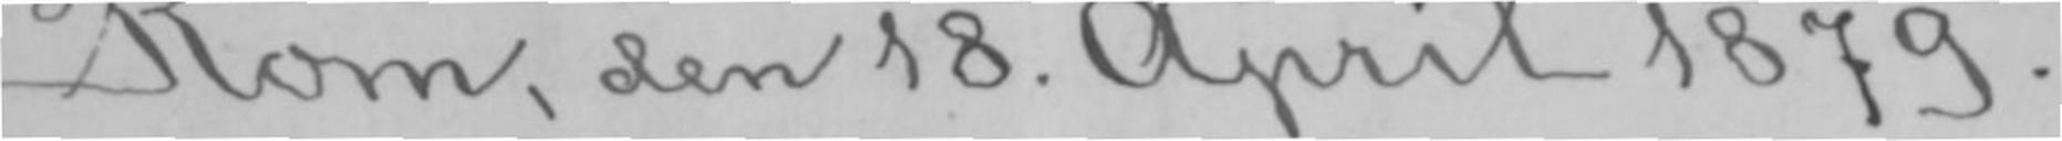Rom, den 18. April 1879.
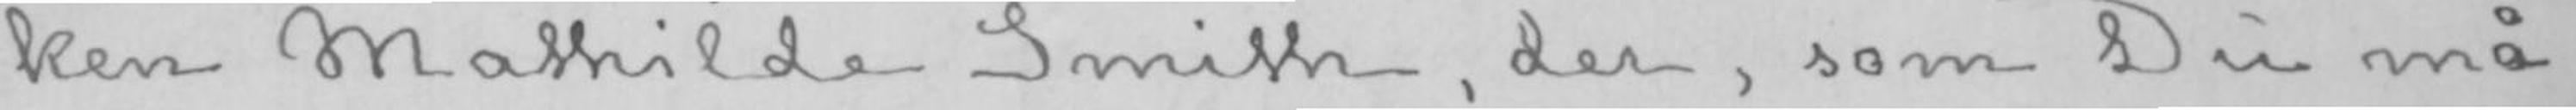ken Mathilde Smith, der, som Du må-
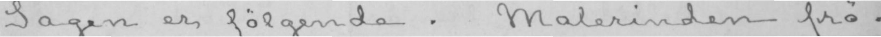Sagen er følgende. Malerinden frø-
